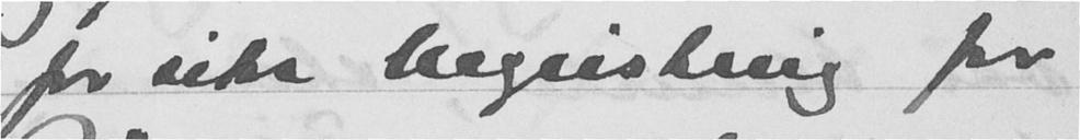for sin begeistning for
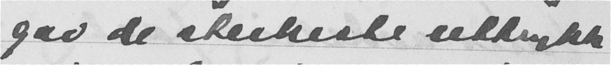og gav de sterkeste uttrykk
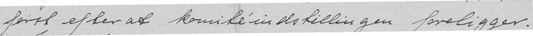først efter at komiteindstillingen foreligger.
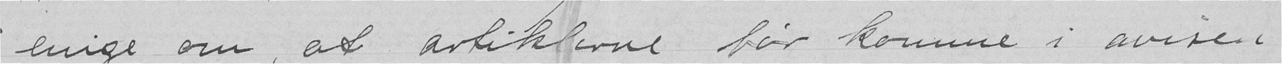enige om, at artiklerne bør komme i avisen
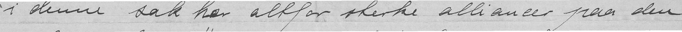i denne sak har altfor sterke alliancer paa den
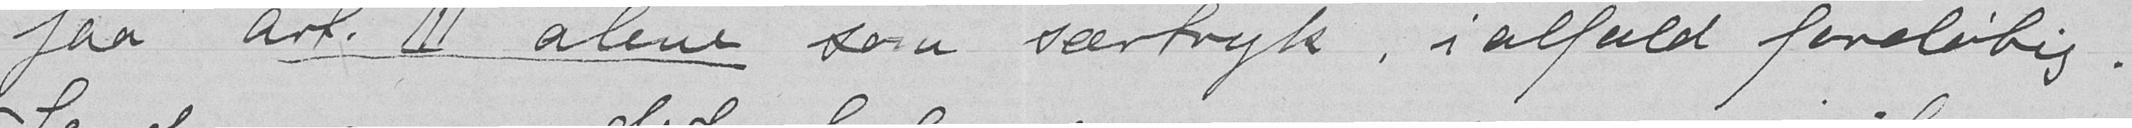faa art. III alene som særtryk, ialfald foreløbig.
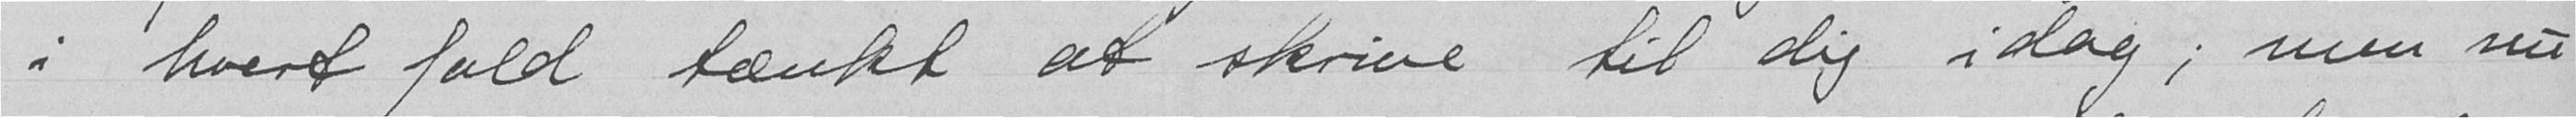i hvert fald tænkt at skrive til dig idag; men nu
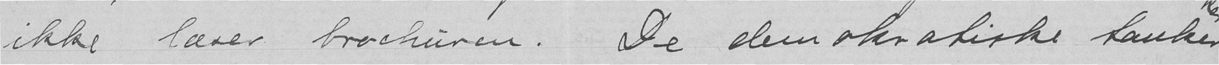ikke læser brochuren. De demokratiske tanker
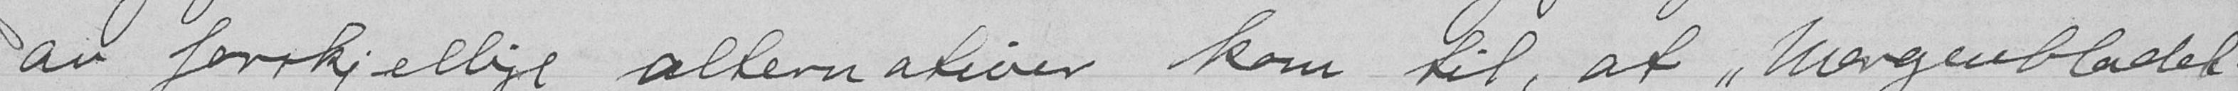av forskjellige alternativer kom til, at Morgenbladet
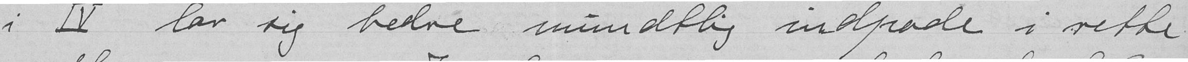i IV lar sig bedre mundtlig indpode i rette
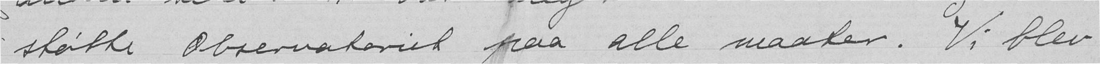støtte Observatoriet paa alle maater. Vi blev
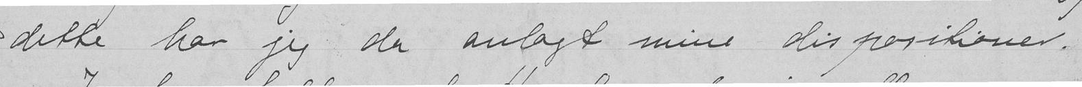dette har jeg da anlagt mine dispositioner.
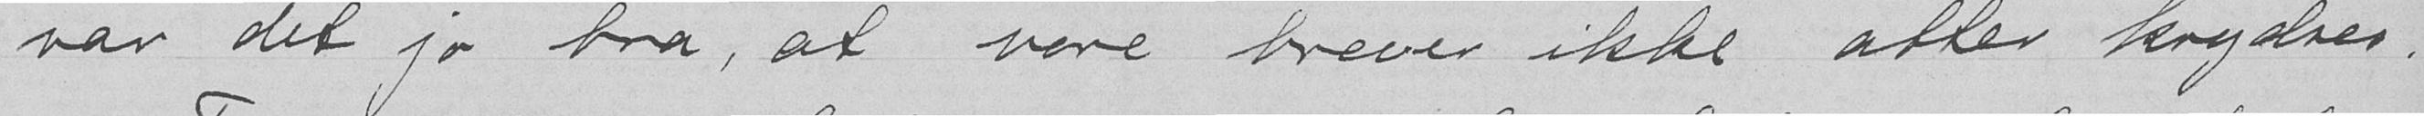var det jo bra, at vore brever ikke atter krydses.
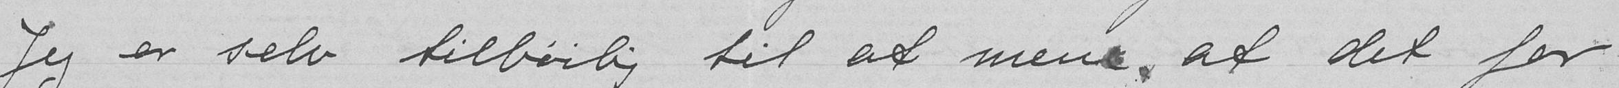Jeg er selv tilbøilig til at mene, at det for
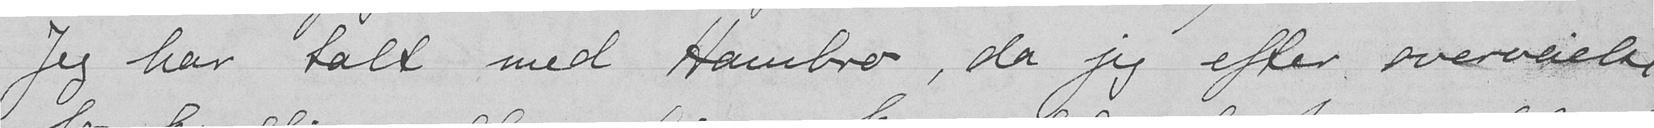Jeg har talt med Hambro, da jeg efter overveielse
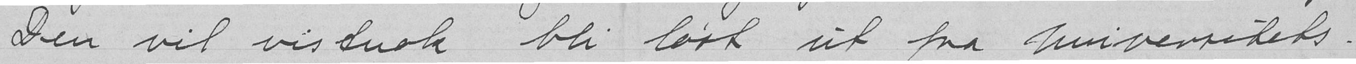Den vil vistnok bli løst ut fra Universitets
